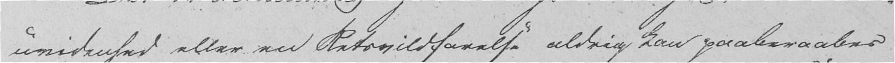uvidenhed eller en Retsvildfarelse aldrig kan paaberaabes
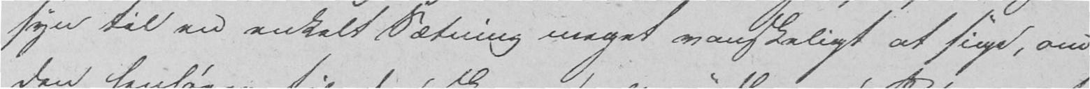syn til en enkelt Sætning meget vanskeligt at sige, om
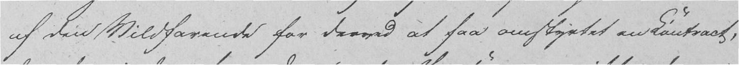af den Vildfarende for derved at faa omstyrtet en Kontract,
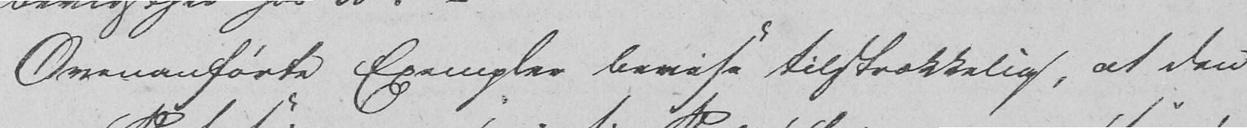Ovenanførte Exempler bevise tilstrækkelig, at den
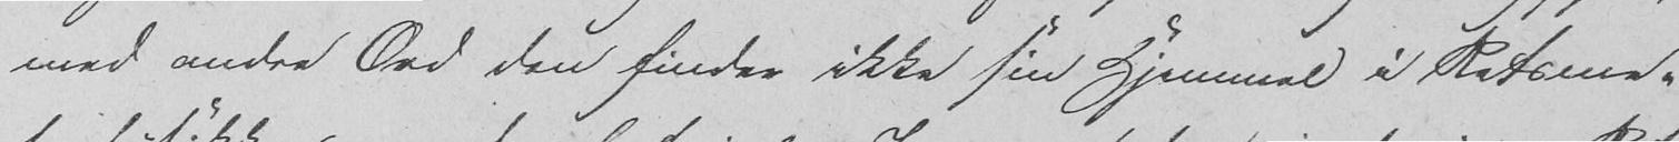med andre Ord den finder ikke sin Hjemmel i Retsme-
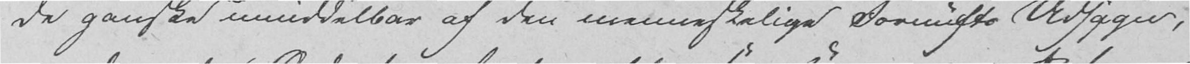de ganske umiddelbar af den menneskelige Fornufts Udsagn,
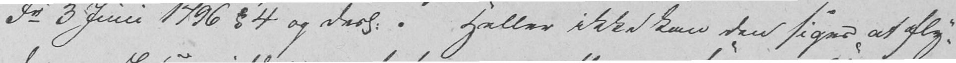Fr 3 Juni 1796 § 4 og desl. Heller ikke kan den siges at fly-
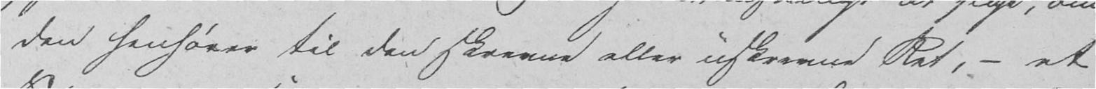den henhører til den skrevne eller uskrevne Ret, – et
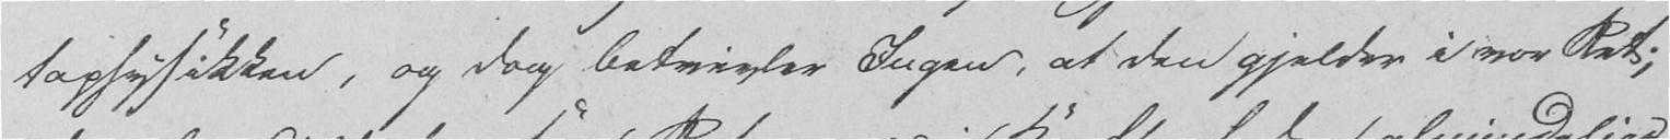taphysikken, og dog betvivler Ingen, at den gjelder i vor Ret;
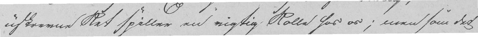uskrevne Ret spiller en vigtig Rolle hos os; men som det
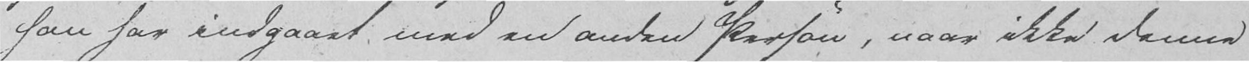han har indgaaet med en anden Person, naar ikke denne
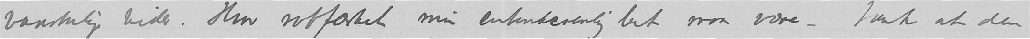vanskelige tider. Hvor rotfæstet min ententevenlighet maa være - tænk at den
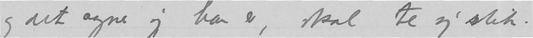og det synes jeg han er, skal te sig slik.
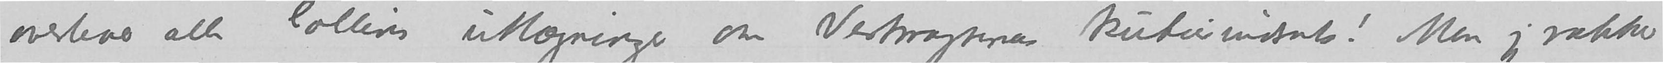overlever alle Collins utlægninger om Vestmagternes kulturindsats! Men jeg rækker
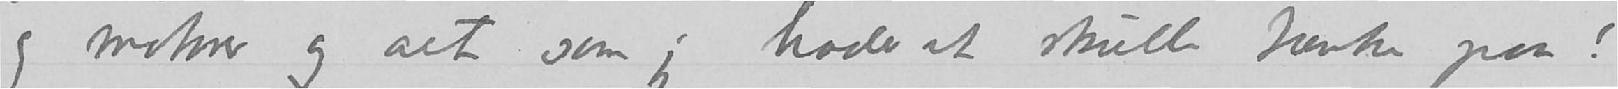og motorer og alt som jeg hader at skulle tænke paa!
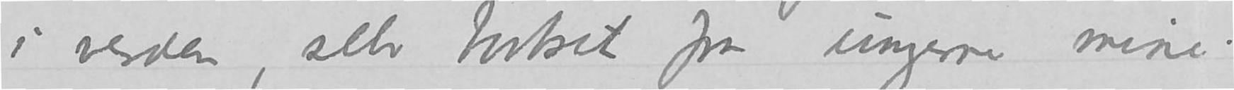i verden, selv bortset fra ungerne mine.
